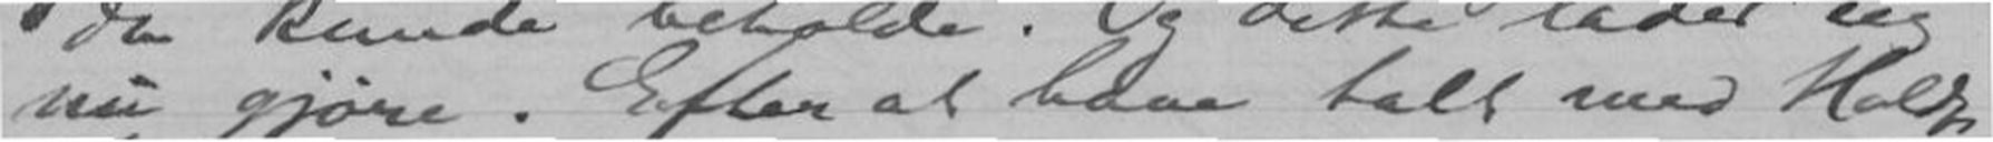nu gjøre. Efter at have talt med Holdts
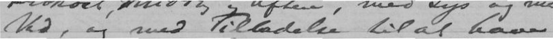Ved, og med Tilladelse til at have
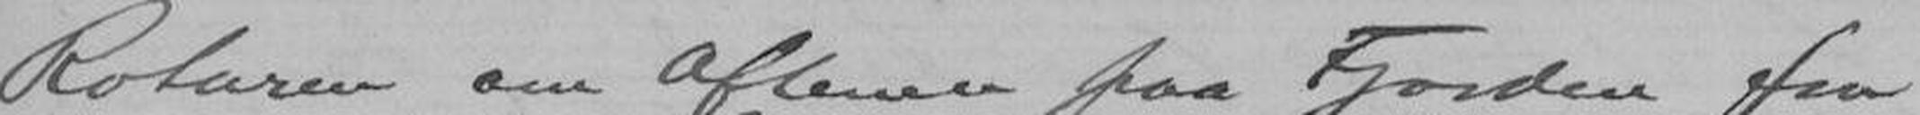Roturen om Aftenen paa Fjorden fra
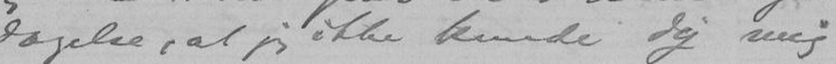dagelse, at jeg ikke kunde dy mig
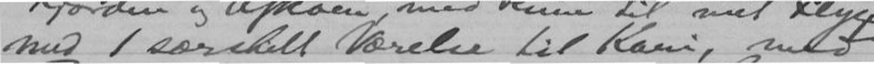med 1 særskilt Værelse til Kari, med
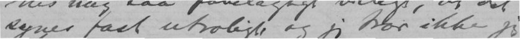synes fast utroligt, og jeg tror ikke jeg
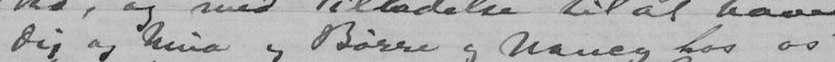Dig og Nina og Børre og Nancy hos os -
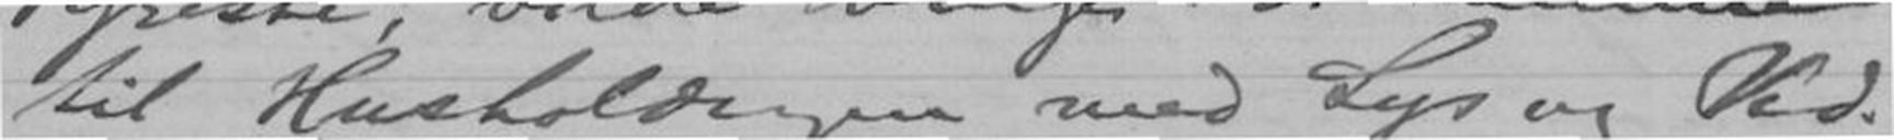til Husholdningen med Lys og ved.
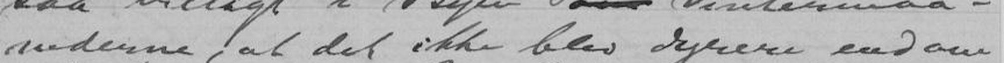nederne, at det ikke blev dyrere end om
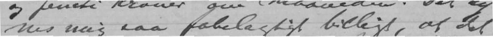nes mig saa fabelagtigt billigt, at det
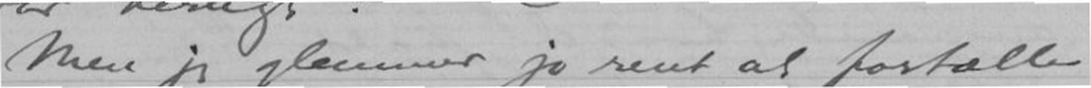Men jeg glemmer jo rent at fortælle
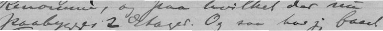paabygges 2 Etager. Og saa har jeg faaet
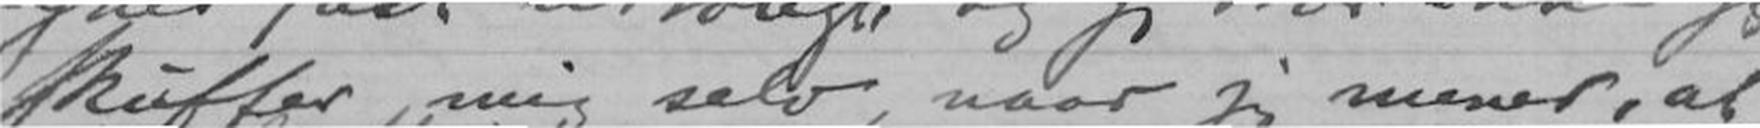skuffer mig selv, naar jeg mener, at
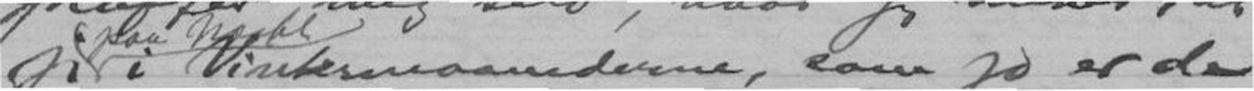jeg i Vintermaanederne, som jo er de
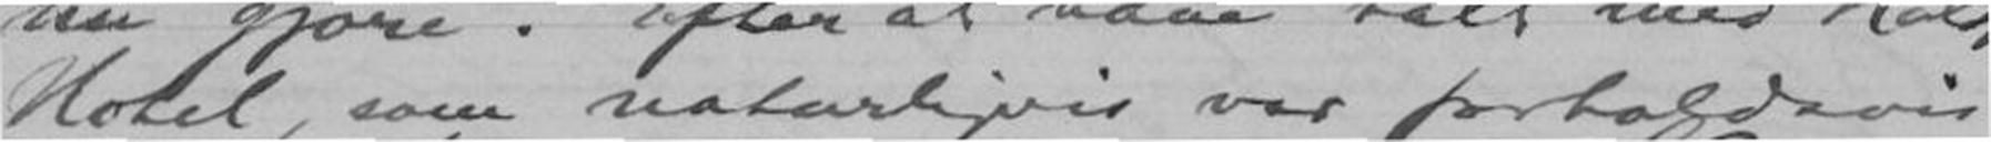Hotel, som naturligvis var forholdsvis
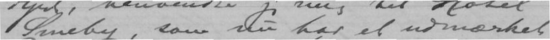Smeby, som nu har et udmærket
